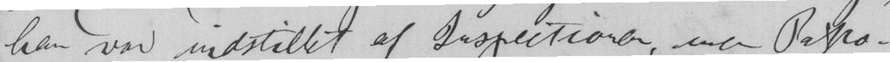han var indstillet af Inspectionen, men Pasto-
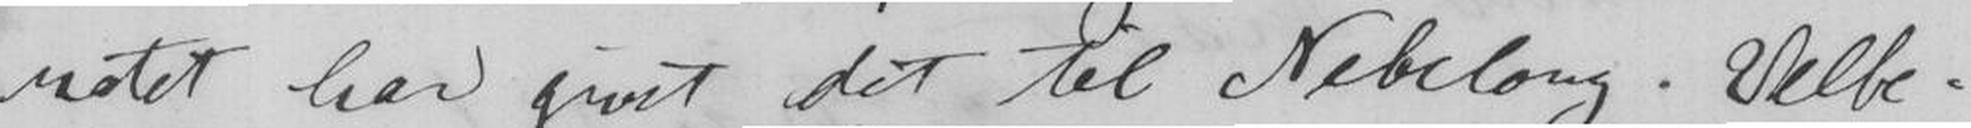ra..tet har givet det til Nebelong. Velbe-
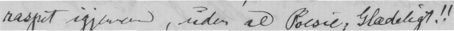raspet igjennem, uden al Poesie; Glædeligt!!
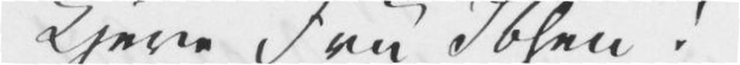Kjere Fru Ibsen!
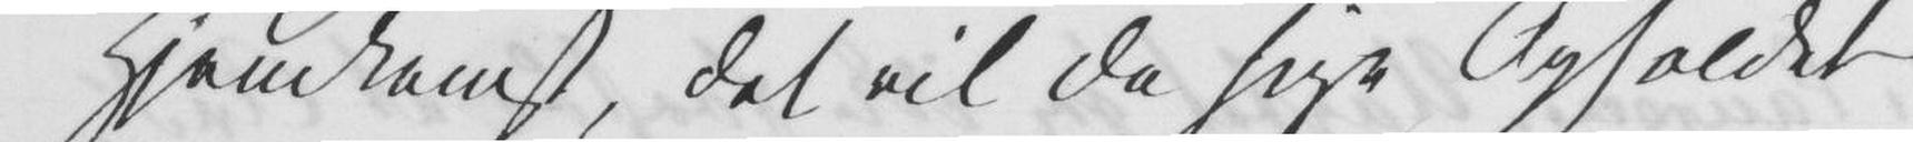Hjemkomst, det vil da sige Opholdet
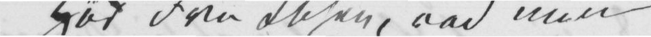Hør Fru Ibsen, ved min
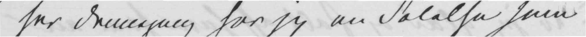her dennegang har jeg en Følelse som
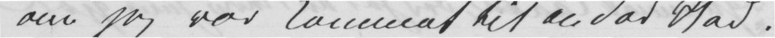om jeg var kommet til en død Stad.
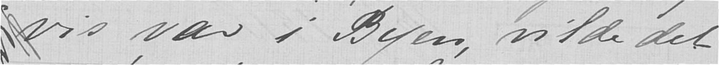vis var i Byen, vilde det
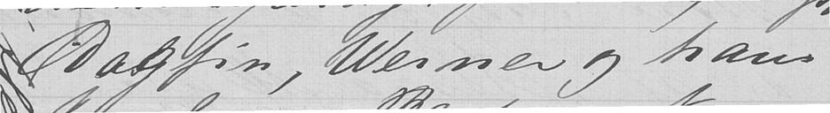Dagfin, Werner og hans
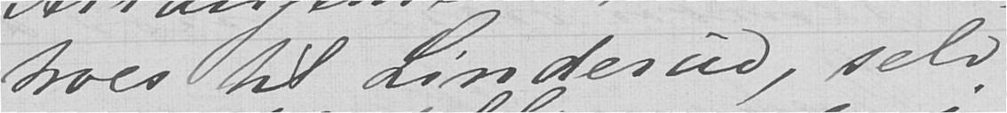troes til Linderud, selv
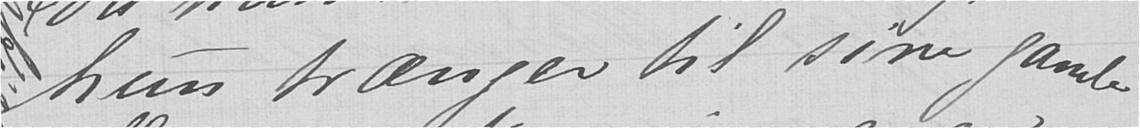hun trænger til sine gamle
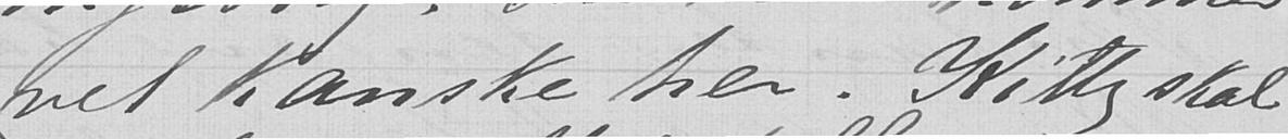vel kanske her. Kitty skal
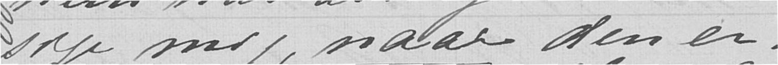sige mig, naar den er.
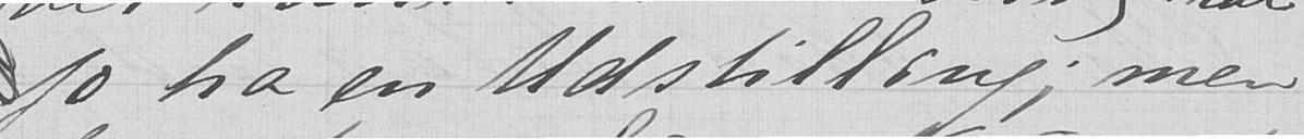jo ha en Udstilling; men
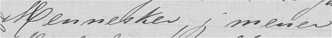Mennesker, jeg mener
